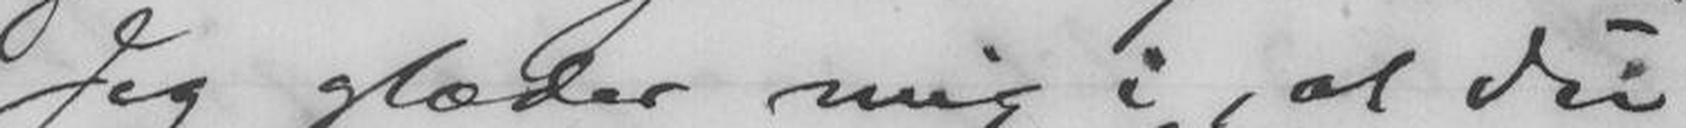Jeg glæder mig i, at Du
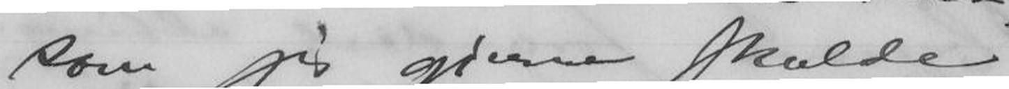som jeg gjerne skulde
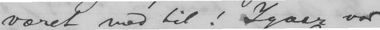været med til! Igaar var
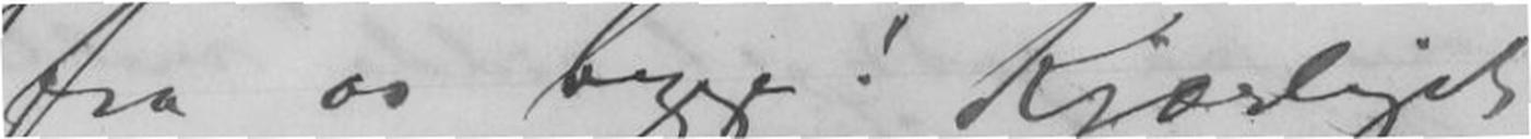fra os begge! Kjærligst
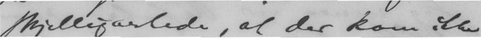skjelligartede, at der kom ikke
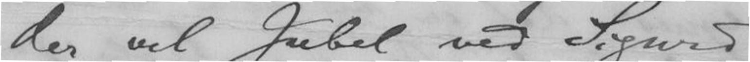der vel Jubel ved Sigurd
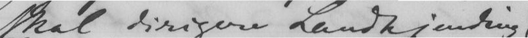skal dirigere Landkjending!
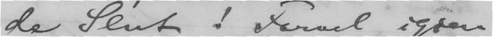de Slut! Farvel igjen
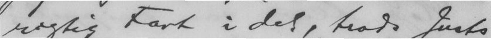rigtig Fart i det, trods Justs
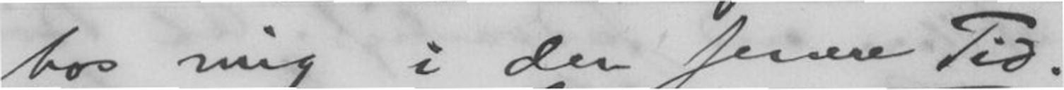hos mig i den senere Tid.
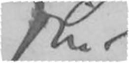Din
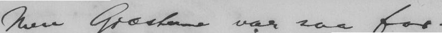Men Gjæstene var saa for-
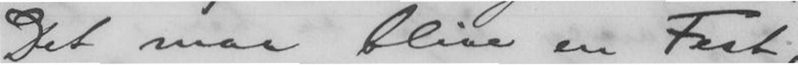Det maa blive en Fest,
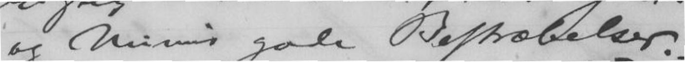og Mimis gode Bestræbelser. -
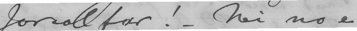Jorsalfar! - Nei no e
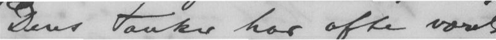Dens tanker har ofte været
